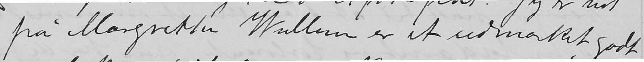på Margrethe Wullum er et udmærket godt
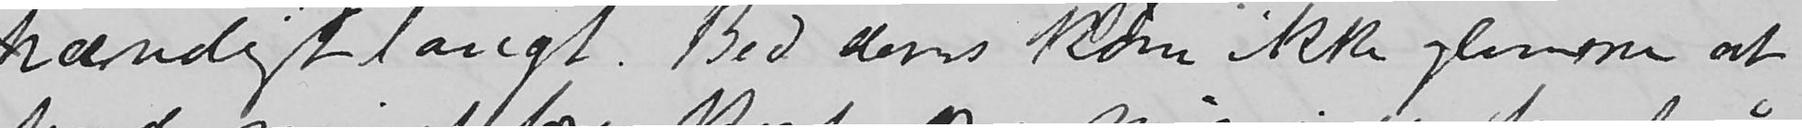hændigt langt. Bed deres kone ikke glemme at
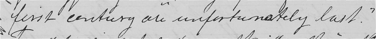first century are unfortunately lost."
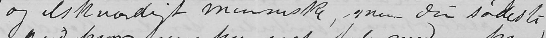og elskværdigt menneske, men du sødeste
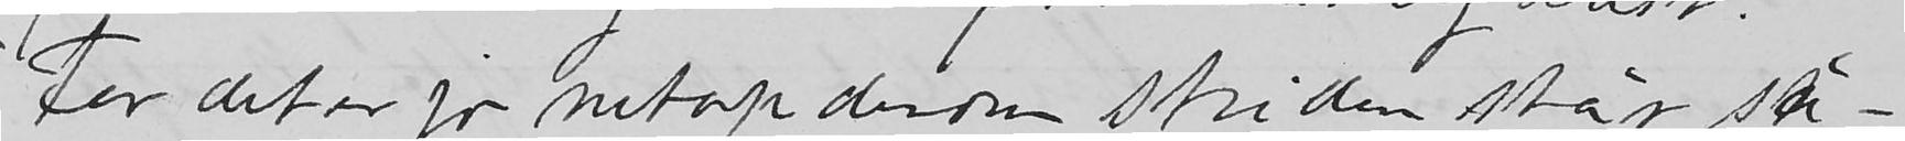For det er jo netorp derom striden står så-
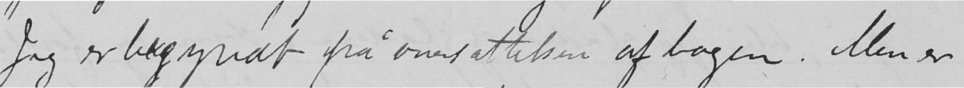Jeg er begyndt på oversættelsen af bogen. Men er
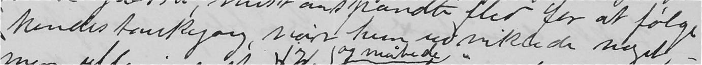hendes tankegang, når hun udviklede noget, -
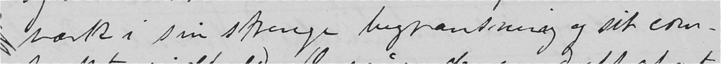værk i sin strenge begrænsning og sit com-
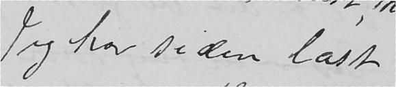Jeg har siden læst
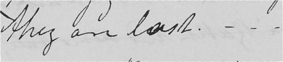they are lost. - - -
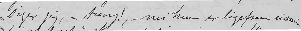siger jeg, - tung!, - nei hun er ligefrem umu-
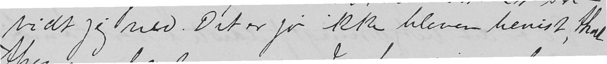vidt jeg ved. Det er jo ikke bleven bevist, that
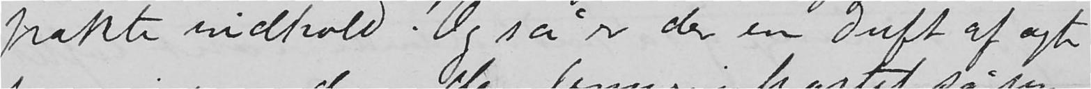pakte indhold. Og så er der en duft af ægte
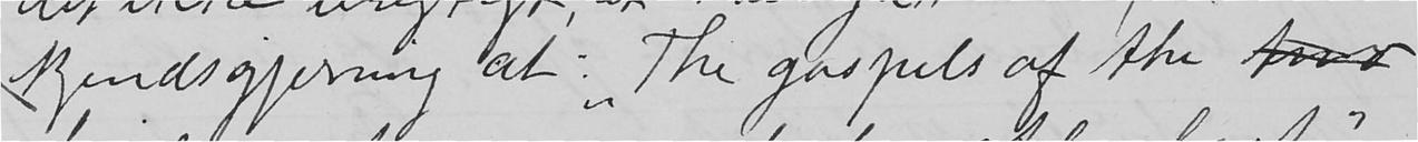kjendsgjerning at: "The gospels of the
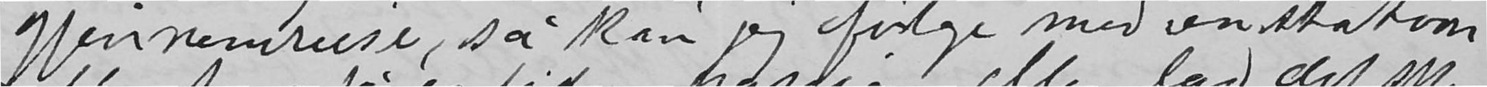gjennemreise, så kan jeg følge med en station
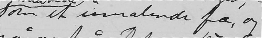som et umælende fæ, og
